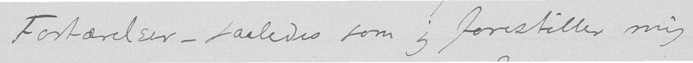Fortærelser - saaledes som jeg forestiller mig
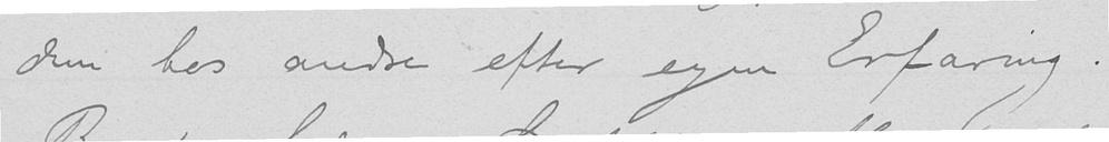dem hos andre efter egen Erfaring.
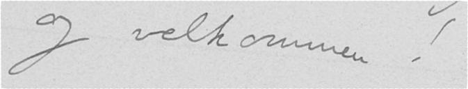og velkommen!
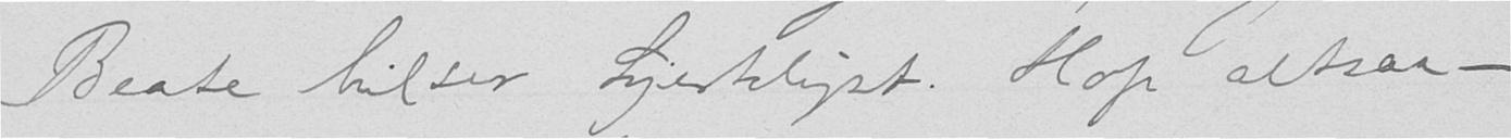Beate hilser hjerteligst. Hop altsaa -
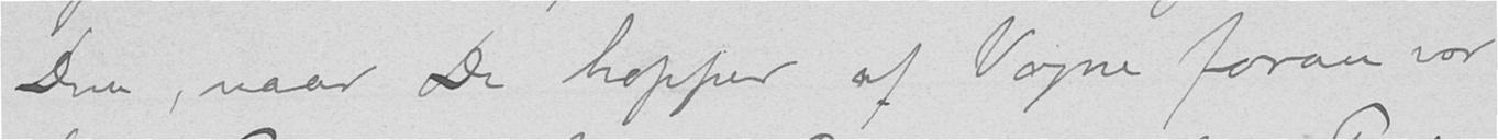Dem, naar De hopper af Vogne foran vor
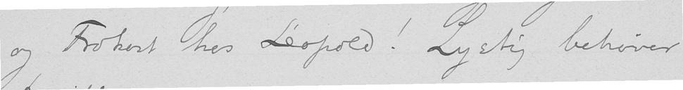og Frokost hos Léopold! Lystig behøver
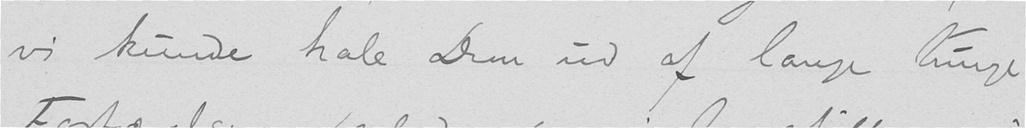vi kunde hale Dem ud af lange tunge
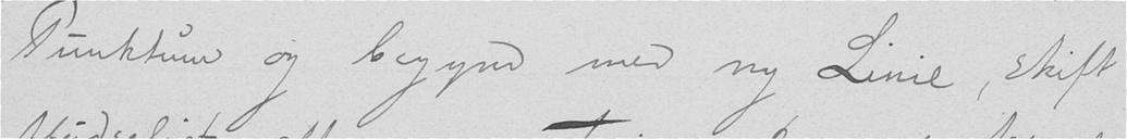Punktum og begynd med ny Linie, skift
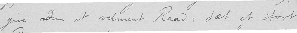give Dem et velment Raad: sæt et stort
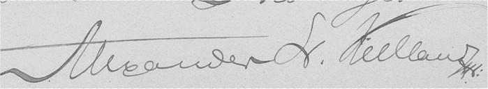Alexander L. Kielland
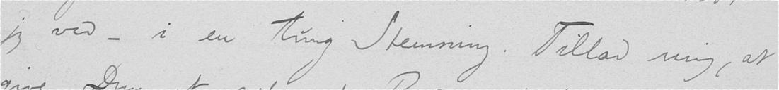jeg ved - i en tung Stemning. Tillad mig, at
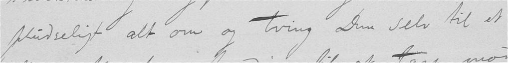pludseligt alt om og tving Dem Selv til et
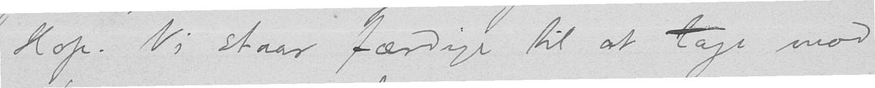Hop. Vi staar færdige til at tage mod
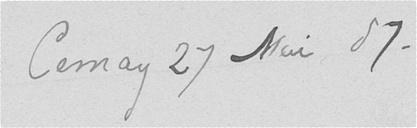Cernay 27 Mai 87.
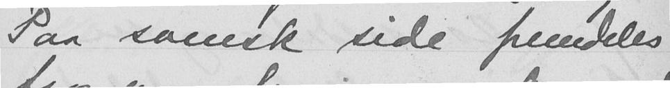Paa svensk side fremdeles
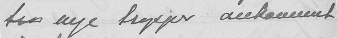tro nye tropper ankommet
